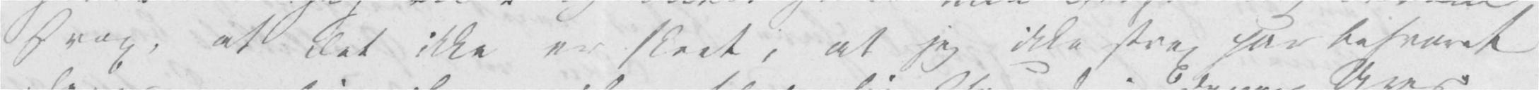strax, at det ikke er skeet, at jeg ikke strax har besvaret
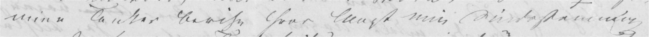mine Tanker bevise hvor langt min Sindsstemning
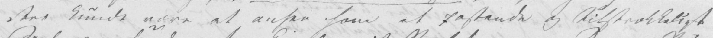kunde være at ansee som et passende og tilstrækkeligt
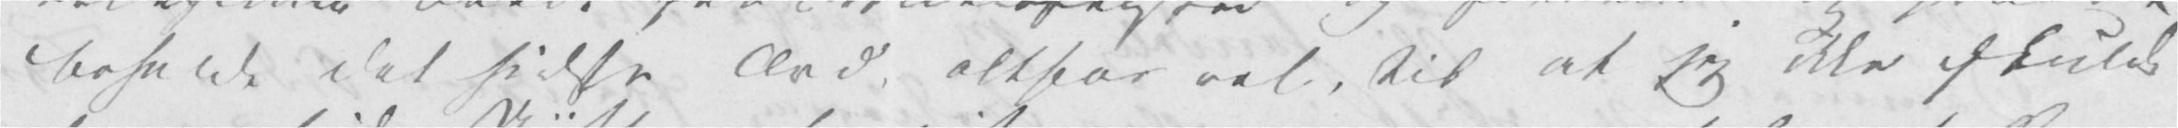beholde det sidste Ord, altfor vel, til at jeg ikke skulde
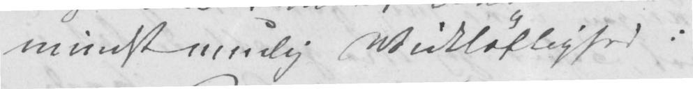mindst muelig Vidtløftighed:
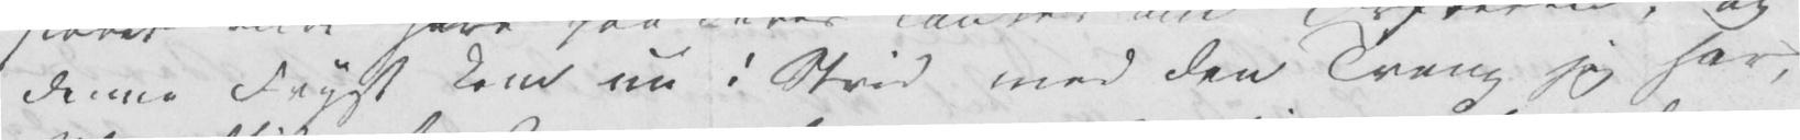denne Frygt kom nu i Strid med den Trang jeg har,
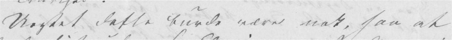Uagtet dette burde være nok, saa at
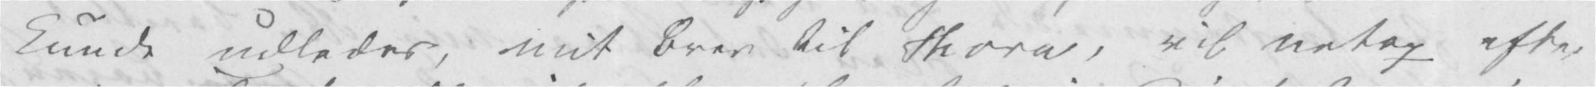kunde udledes, mit Brev til Thora, vil netop efter
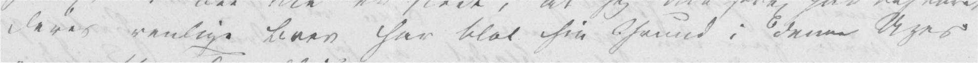Deres venlige Brev har blot sin Grund i denne Uges
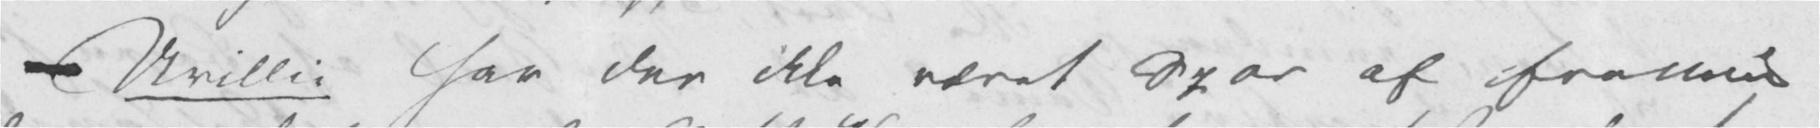– Uvillie har der ikke været Spor af ifra min
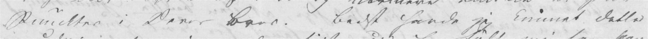Punckter i Deres Brev. Bedst havde jeg kunnet dette
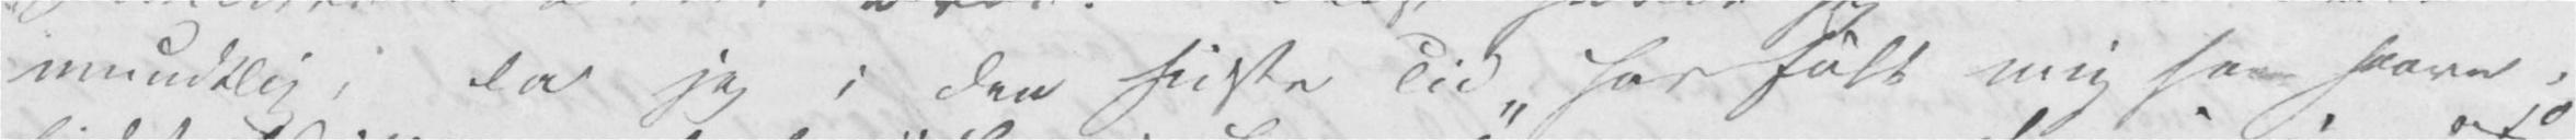mundtlig, da jeg i den sidste Tid har følt mig saa saare
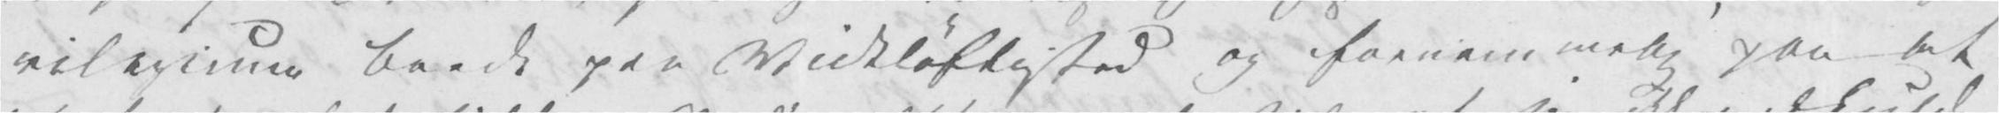vilegium baade paa Vidtløftighed og fornemmelig paa at
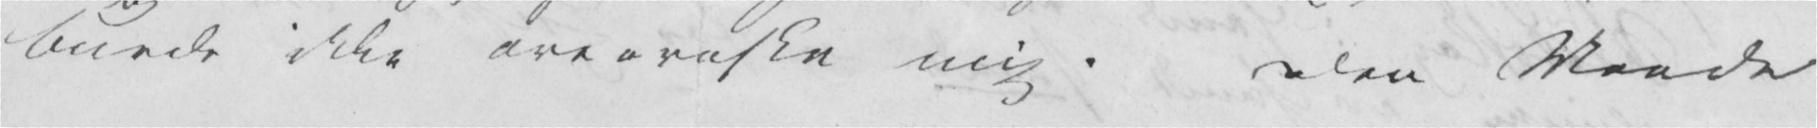burde ikke overraske mig. Den Maade
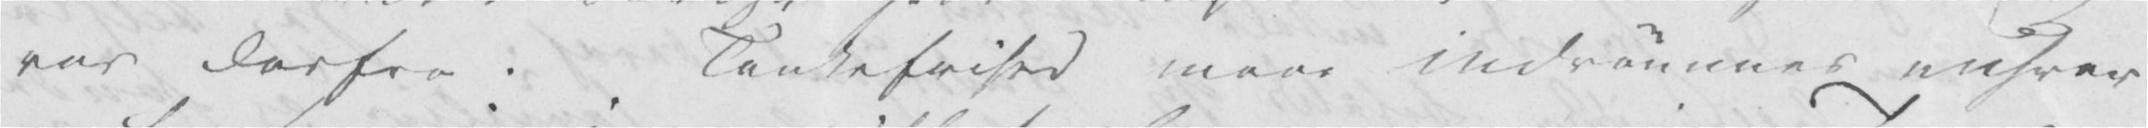var derfra. Tankefrihed maae indrømmes enhver
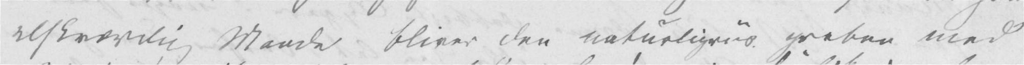elskværdig Maade bliver den naturligviis greben med
